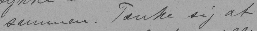sammen. Tænke sig at
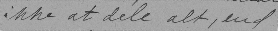ikke at dele alt, end
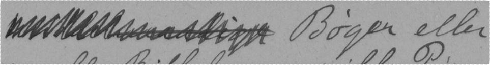Bøger eller
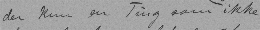der kun en Ting som ikke
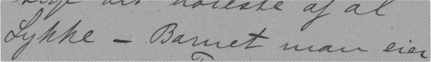Lykke - Barnet man eier
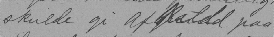skulde gi Afkaldd paa
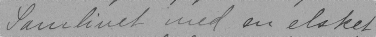Samlivet med en elsket
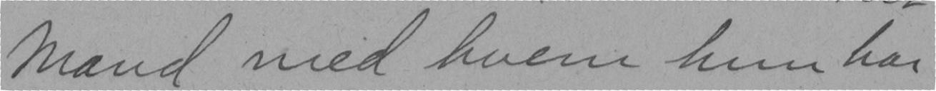mand med hvem hun har
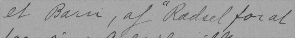et Barn, af "Rædsel for at
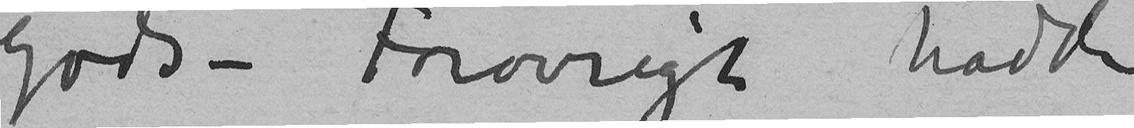Gods – Forøvrigt hadde
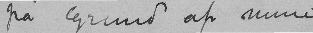på Grund af mine
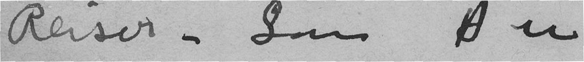Reiser – Som Du
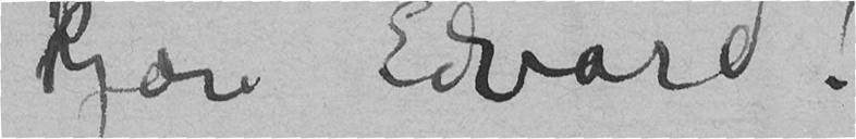Kjære Edvard!
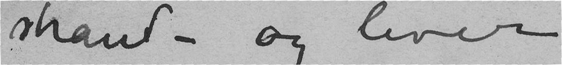strand – og lever
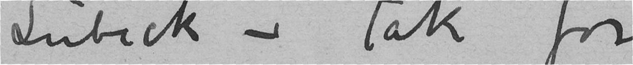Lübeck – Tak for
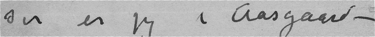ser er jeg i Aasgaard-
